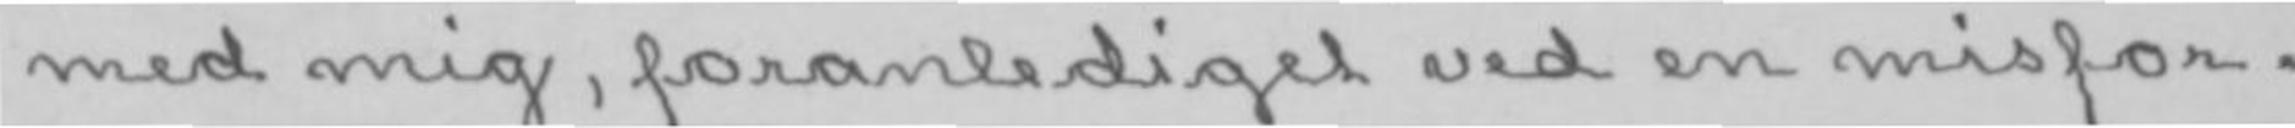med mig, foranlediget ved en misfor-
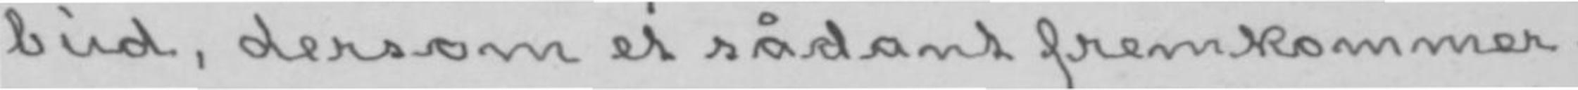bud, dersom et sådant fremkommer.
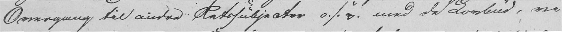Overgang til andre Retssubjecter o. s. v. med de Lovbud, vi
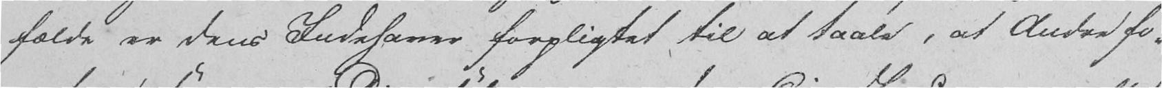fælde er Dens Indehaver forpligtet til at taale, at Andre fo-
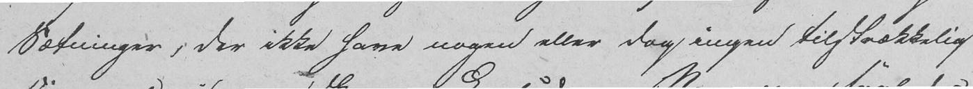Sætninger, der ikke have nogen eller dog ingen tilstrækkelig
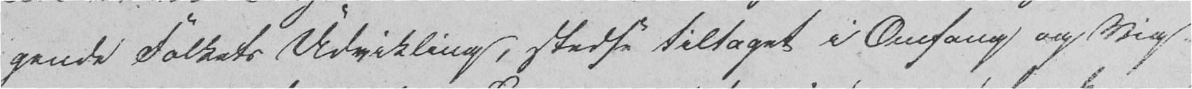gende Folkets Udvikling, stedse tiltaget i Omfang og Vig-
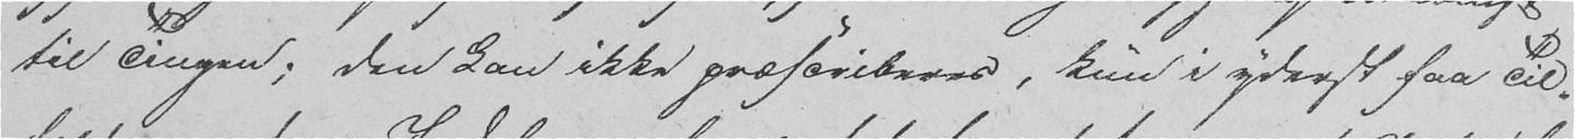til Tingen; den kan ikke præscriberes, kun i yderst faa Til-
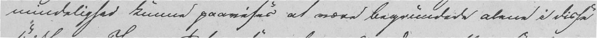mindelighed kunne paavises at være Begrundede alene i disse
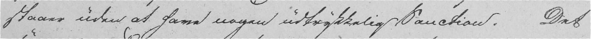staaer uden at have nogen udtrykkelig Sanction. Det
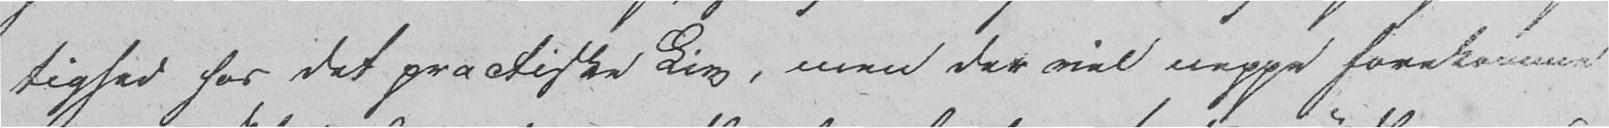tighed for det practiske Liv, men der vil neppe forekomme
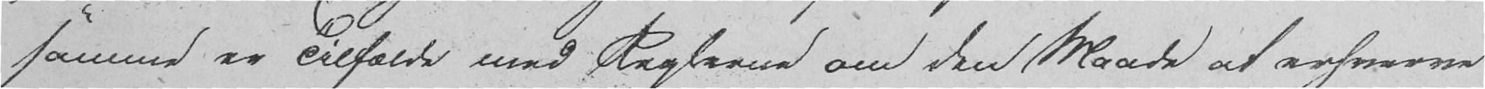samme er Tilfælde med Reglerne om den Maade at erhverve
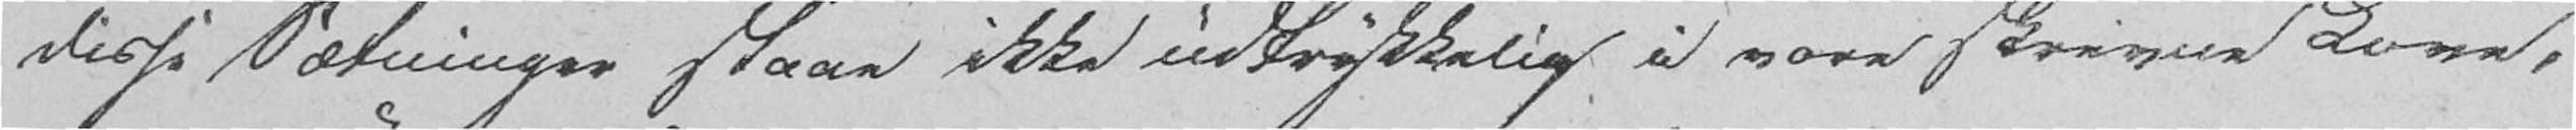disse Sætninger staar ikke udtrykkelig i vore skrevne Love,
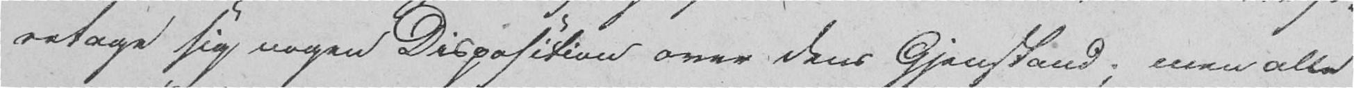retage sig nogen Disposition over dens Gjenstand; men alle
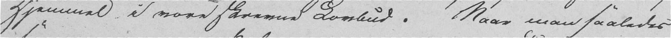Hjemmel i vore skrevne Lovbud. Naar man saaledes
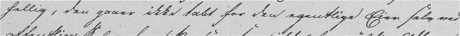hellig, den gaaer ikke tabt for den egentlige Eier selv ved
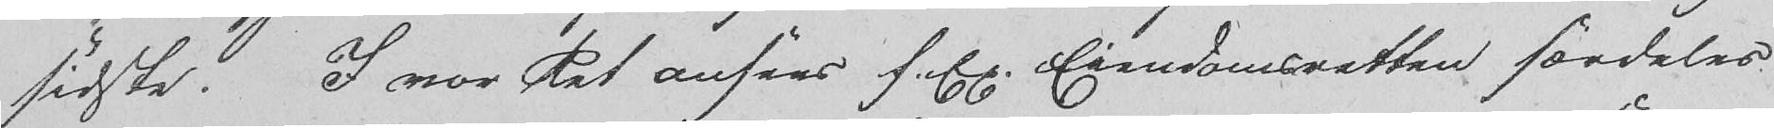sidste. I vor Ret ansees f. Ex. Eiendomsretten særdeles
